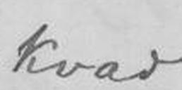Kvad
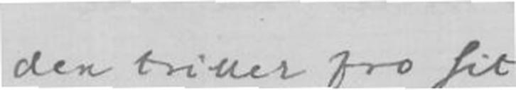den triller paa sit
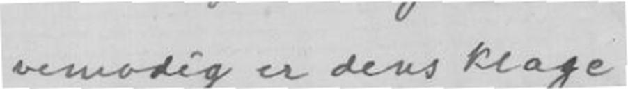vemodig er dens klage
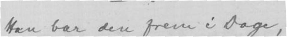Han bar den frem i Dage,
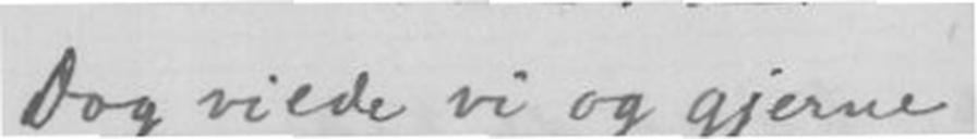Dog vilde vi og gjerne
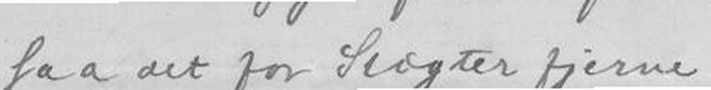saa det for Slægter fjerne
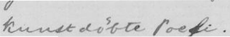kunstdøbte Poesi.
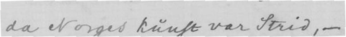da Norges kunst var Strid, -
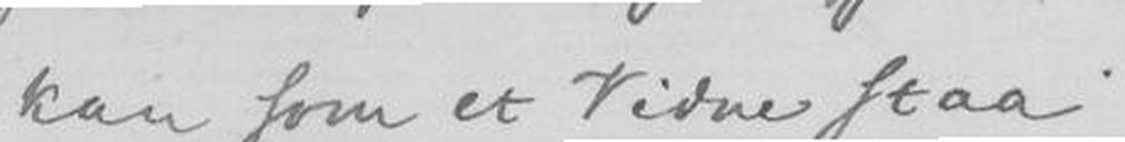kan som et Vidne staa
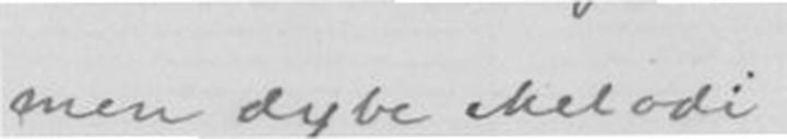men dybe Melodi
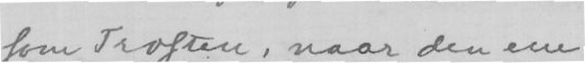som Trosten, naar den ene
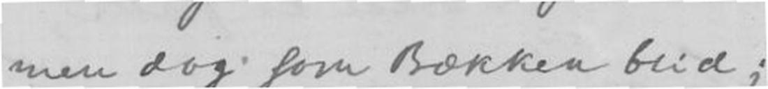men dog som Bækken blid;
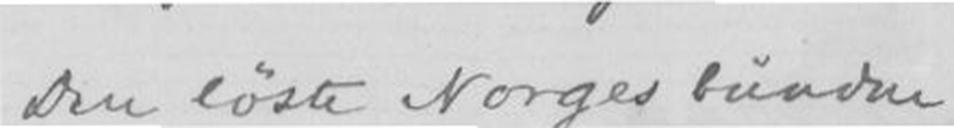Den løste Norges bundne
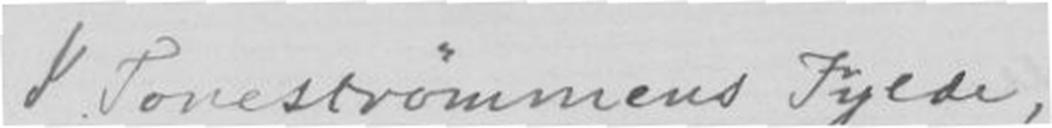I Tonestrømmens Fylde,
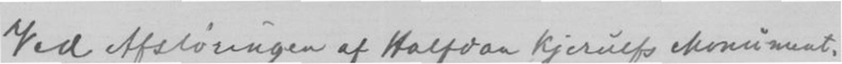Ved Afsløringen af Halfdan Kjerulfs Monument
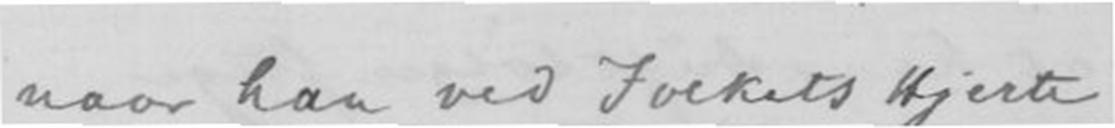naar han ved Folkets Hjerte
